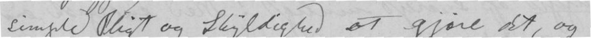simple Pligt og Skyldighed at gjøre det, og
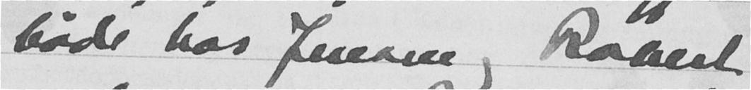både hos Jenann, og Robert
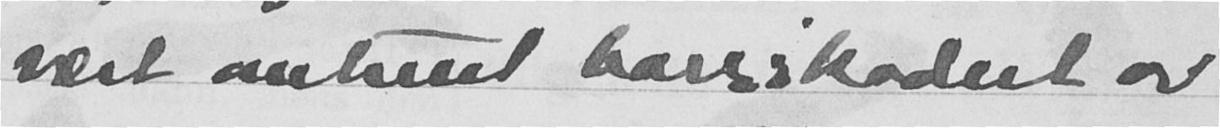vært omtrent barrikadert av
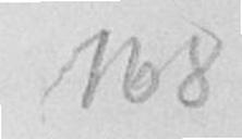168
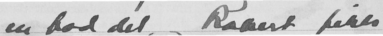en bod det. Robert fikk
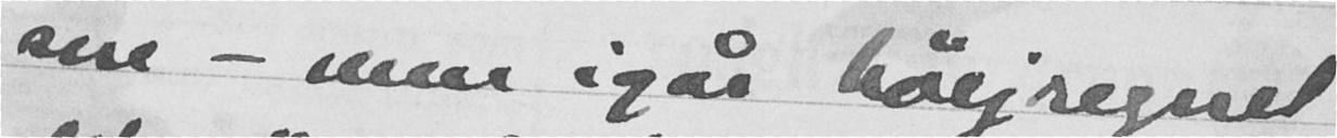sne - men igår høyregnet
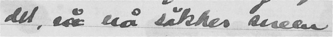det, så nå søkker sneen
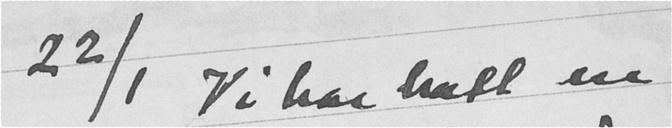22/1 Vi har hatt en
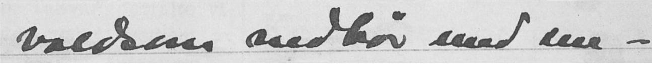voldsom nedbør med sne -
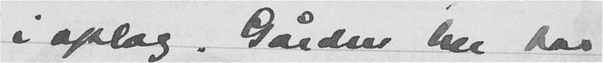i oplag. Gården her har
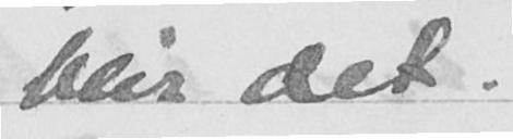blir det.
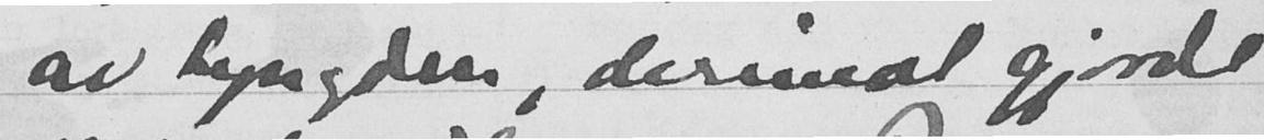av tyngden, derimot gjorde
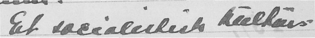Et socialistisk kultur-
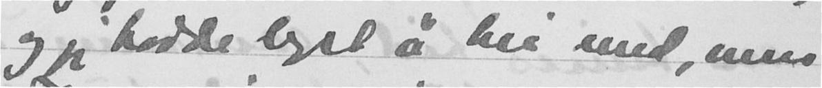og jeg hadde lyst å bli med, men
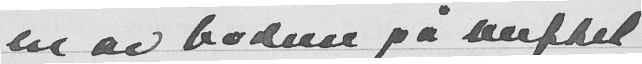en av bodene på verftet
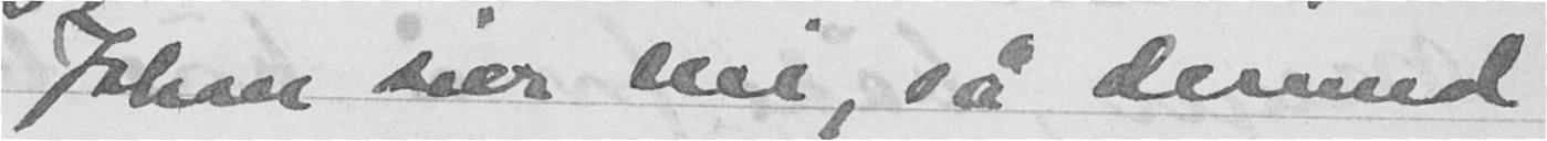Johan sier nei, så dermed
